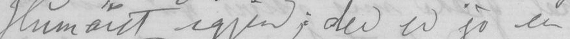Humøret igjen, der er jo en
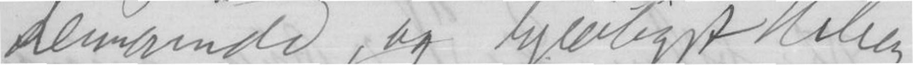dennerinde hjerteligt Hilsen
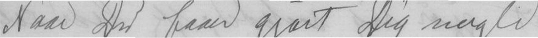Naar Du faaer gjort Dig nogle
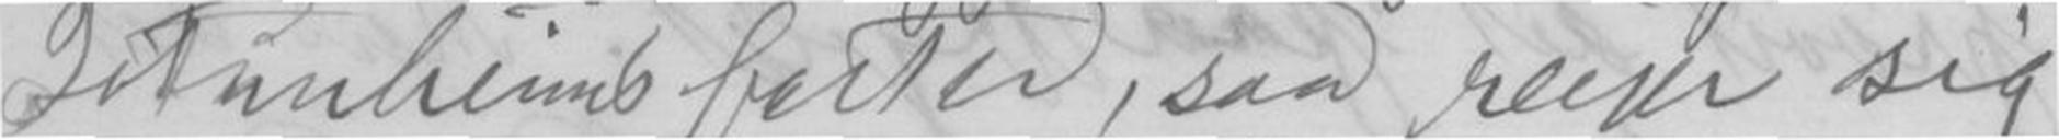Jotunheimsfarter saa reiser sig
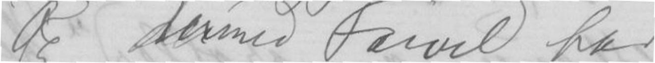Og dermed Farvel for
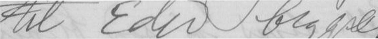til Eder begge
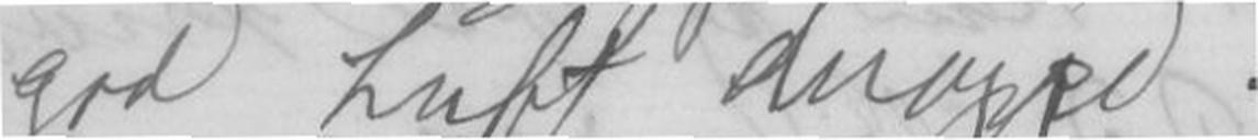god Luft deroppe.
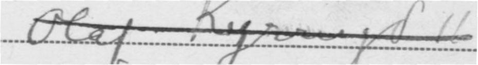Olaf Kyrresgd 11
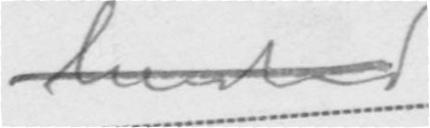Hersted
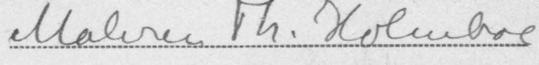Maleren Th. Holmboe
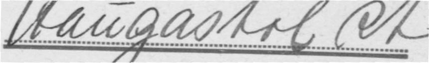Haugastøl st
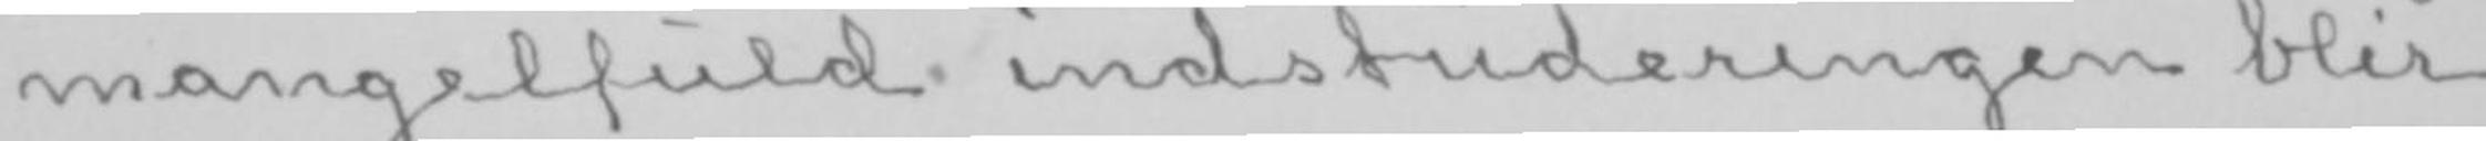mangelfuld indstuderingen blir
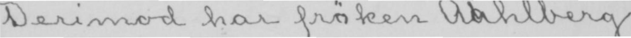Derimod har frøken Ahahlberg
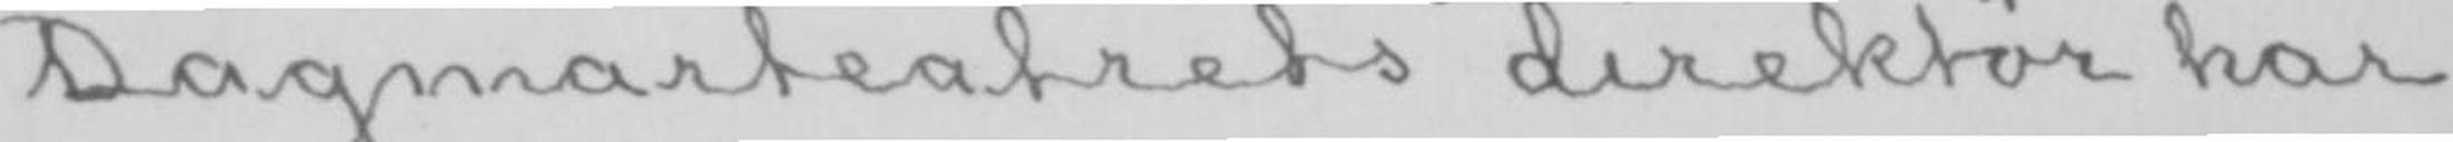Dagmarteatrets direktør har
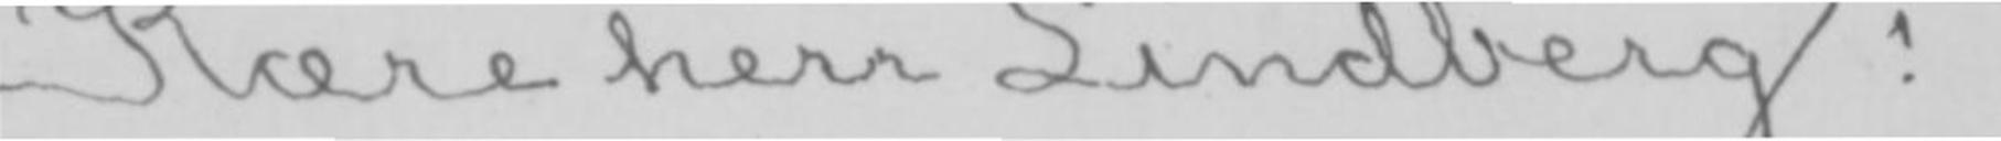Kære herr Lindberg!
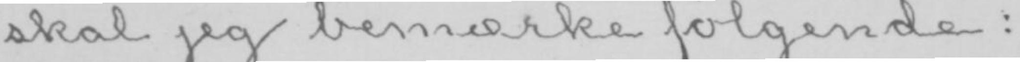skal jeg bemærke følgende:
